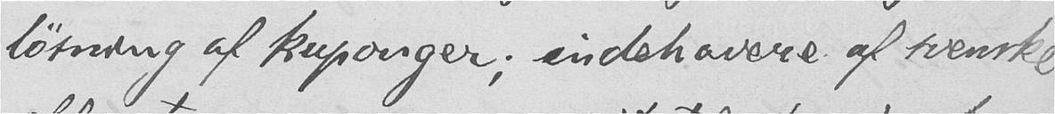løsning af kuponger; indehavere af svenske
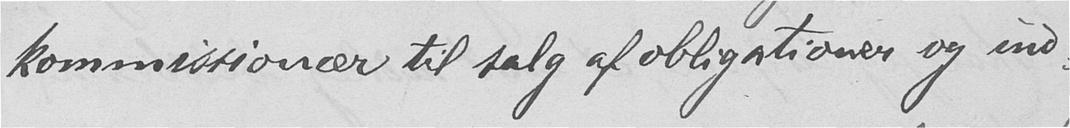kommissionær til salg af obligationer og ind-
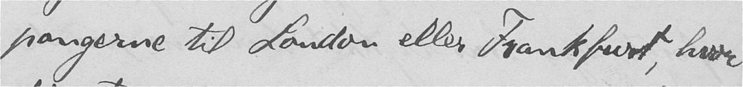pongerne til London eller Frankfurt, hvor
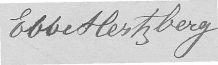Ebbe Hertzberg
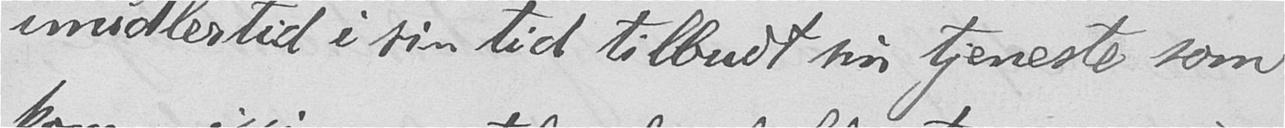imidlertid i sin tid tilbudt sin tjeneste som
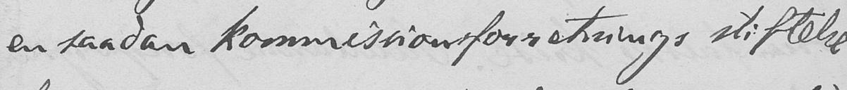en saadan kommissionsforretnings stiftelse
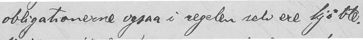obligationerne ogsaa i regelen selv ere kjøbte.
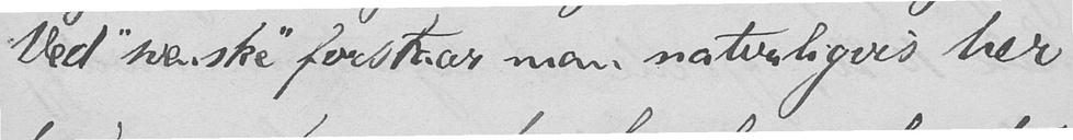Ved "svenske" forstaar man naturligvis her
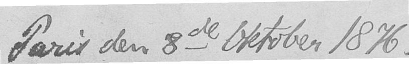Paris den 8de Oktober 1876.
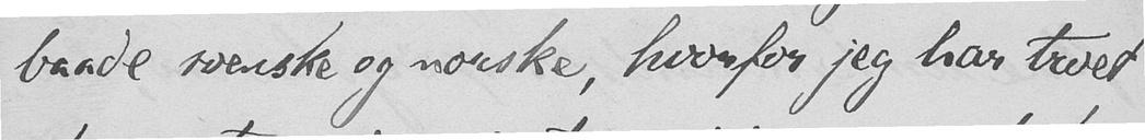baade svenske og norske, hvorfor jeg har troet
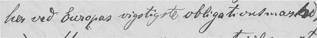her ved Europas vigtigste obligationsmarked,
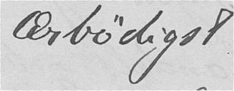Ærbødigst
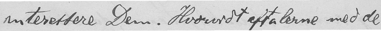interessere Dem. Hvorvidt aftalerne med de
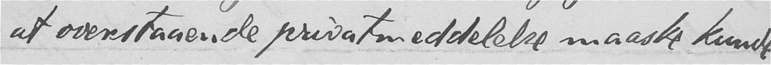at ovenstaaende privatmeddelelse maaske kunde
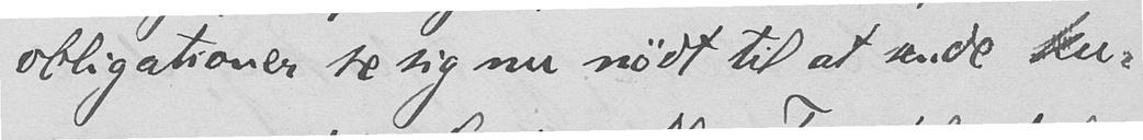obligationer se sig nu nødt til at sende ku-
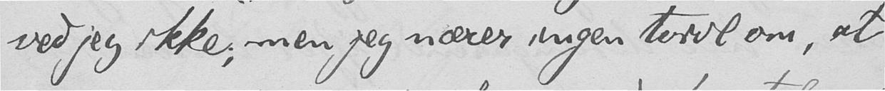ved jeg ikke; men jeg nærer ingen tvivl om, at
